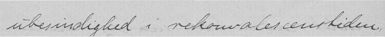ubesindighed i rekonvalescenstiden,
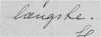længste.
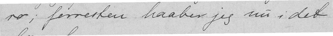ro; forresten haaber jeg nu i det
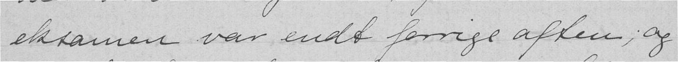eksamen var endt forrige aften; og
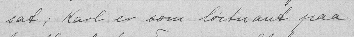sat; Karl er som løitnant paa
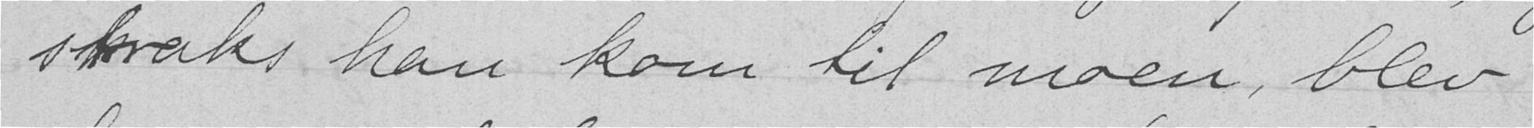straks han kom til moen, blev
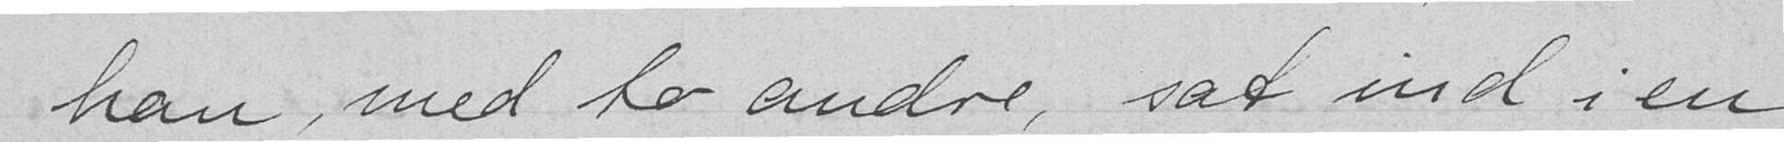han, med to andre, sat ind i en
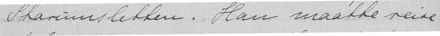Starumsletten. Han maatte reise
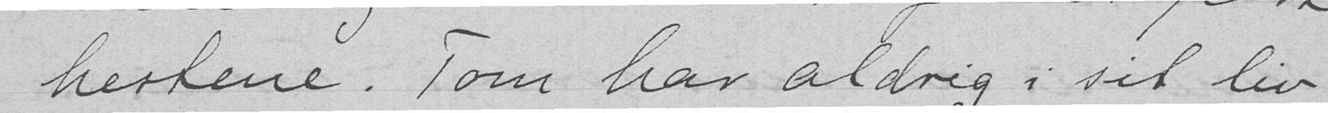hestene. Tom har aldrig i sit liv
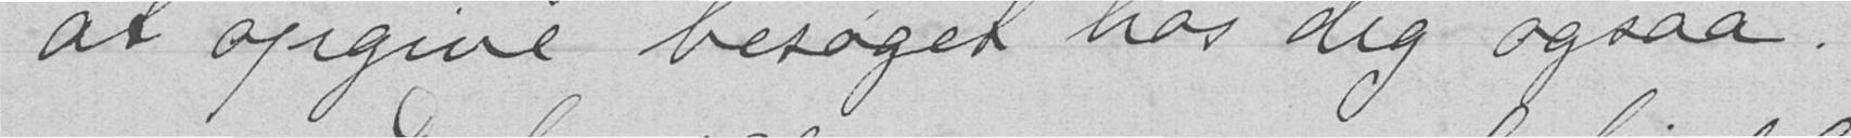at opgive besøget hos dig ogsaa.
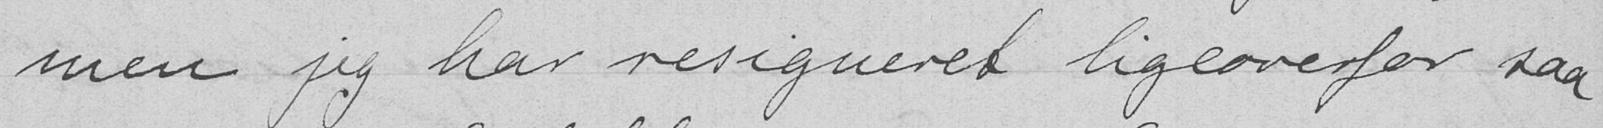men jeg har resigneret ligeoverfor saa
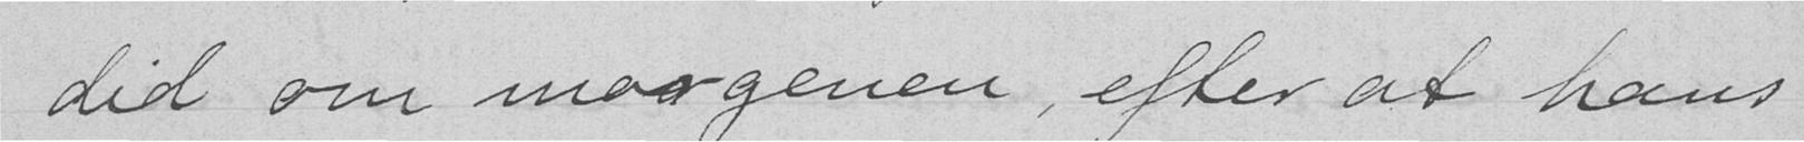did om morgenen, efter at hans
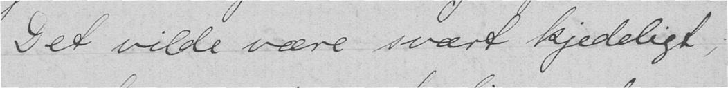Det vilde være svært kjedeligt,
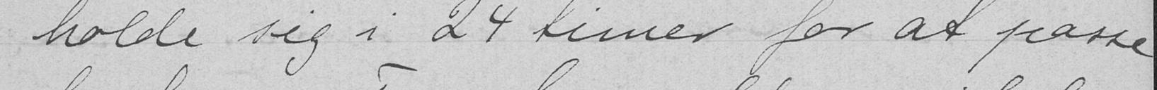24 timer for at passe
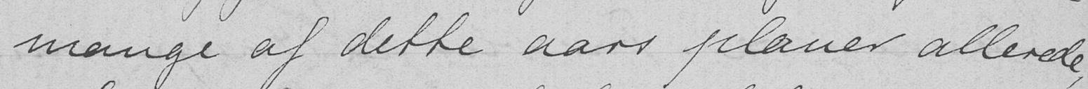mange af dette aars planer allerede,
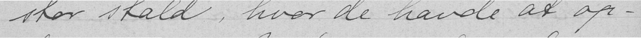stor stald, hvor de havde at op-
